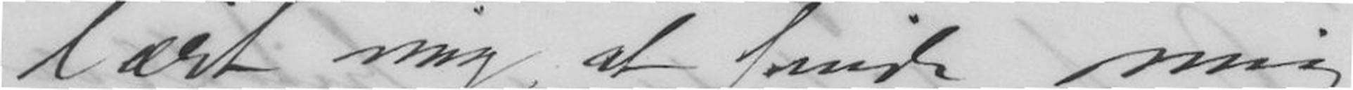lært mig at finde mig
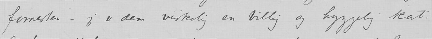forresten – jeg er dem virkelig en billig og hyggelig kat.
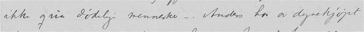ikke qua dødelige mennesker –. Anders har av dyrekjøpt
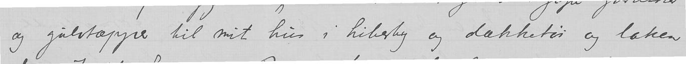og gulvtæpper til mit hus i Liberty og dækketøi og laken
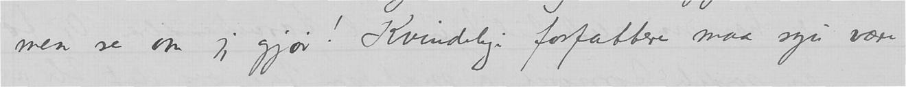men se om jeg gjør! Kvindelige forfattere maa sgu være
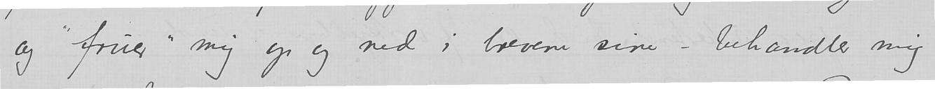og «fruer» mig op og ned i brevene sine – behandler mig
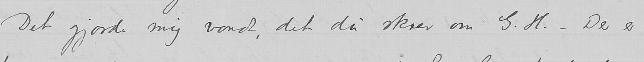Det gjorde mig vondt, det du skrev om G.H. – Der er
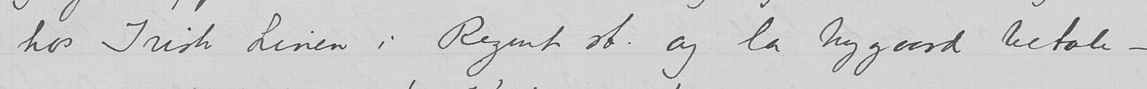hos Irish Linen i Regent st. og la Nygaard betale –
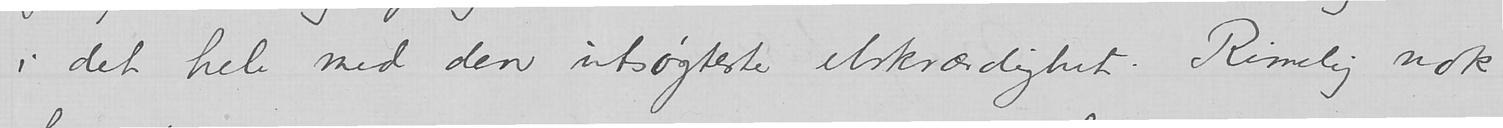i det hele med den utsøgteste elskværdighet. Rimelig nok
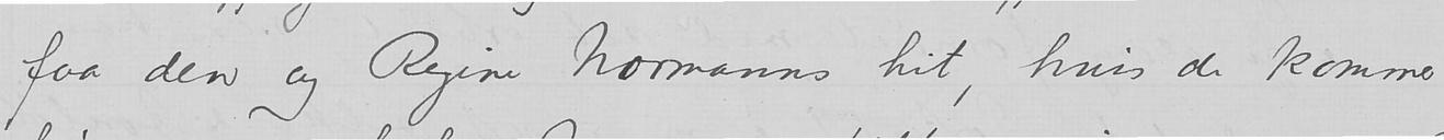faa den og Regine Normanns hit, hvis de kommer
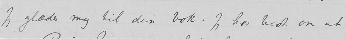Jeg glæder mig til din bok. Jeg har bedt om at
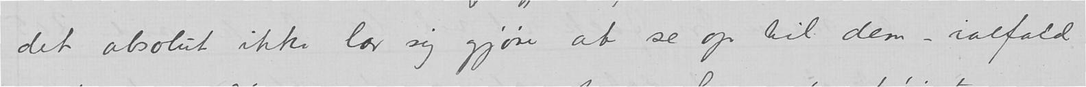det absolut ikke lar sig gjøre at se op til dem – ialfald
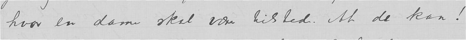hvor en same skal være tilstede. At de kan!
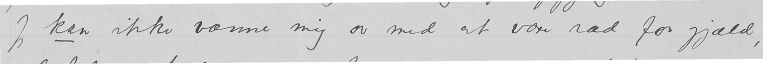Jeg kan ikke vænne mig av med at være ræd for gjæld,
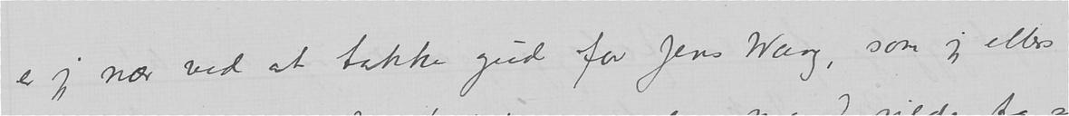er jeg nær ved at takke gud for Jens Wang som jeg ellers
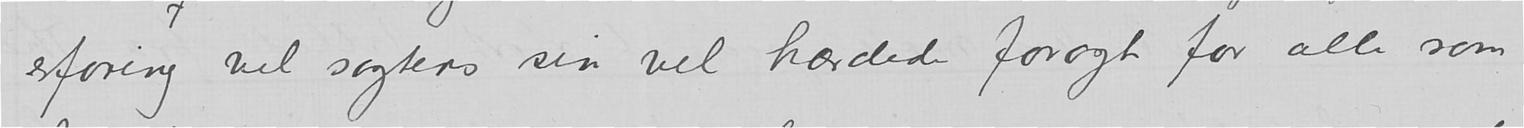erfaring vel sagtens sin vel hærdede foragt for alle som
